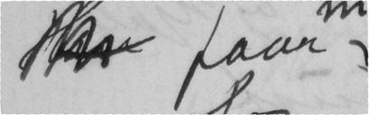faar
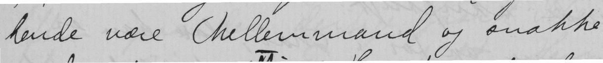hende være Mellemmand og snakke
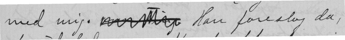med mig.  Han foreslog da,
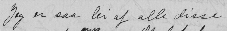Jeg er saa lei af alle disse
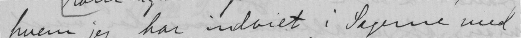hvem jeg har indviet i Sagerne med
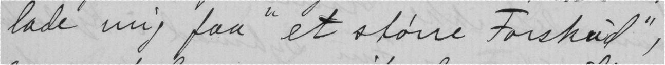lade mig faa "et større Forskud",
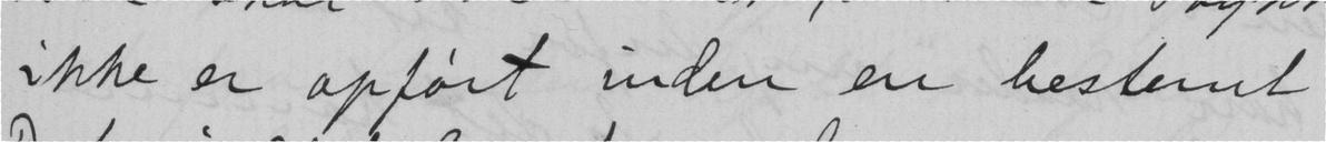ikke er optørt inden en bestemt
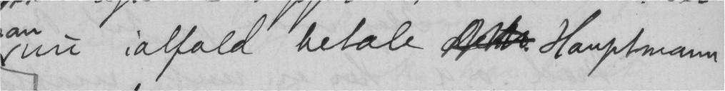nu ialfald betale  Hauptmann
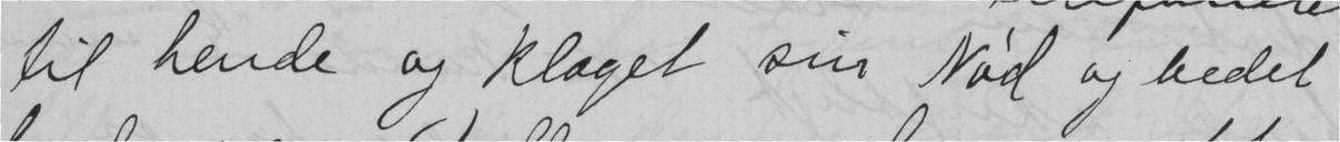til hende og klaget sin Nød og bedet
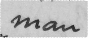man
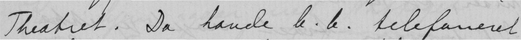Theatret. Da havde b. b. telefoneret
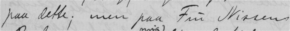paa dette; men paa Fru Nissens
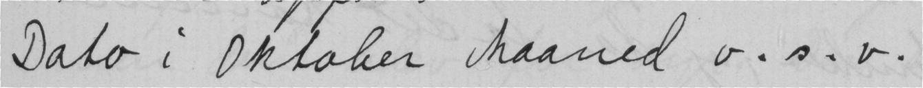Dato i Oktober Maaned o. s. v.
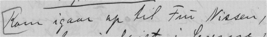Kom igaar op til Fru Nissen,
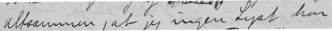altsammen, at jeg ingen Lyst har
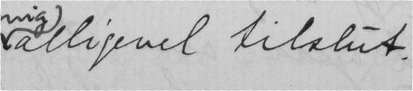alligevel tilslut.
